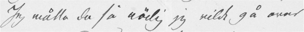Jeg måtte da så nødig jeg vilde gå over
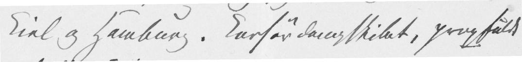Kiel og Hamburg. Korsørdampskibet, propfuldt
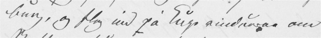burg, og slog ind på Kupevinduerne om
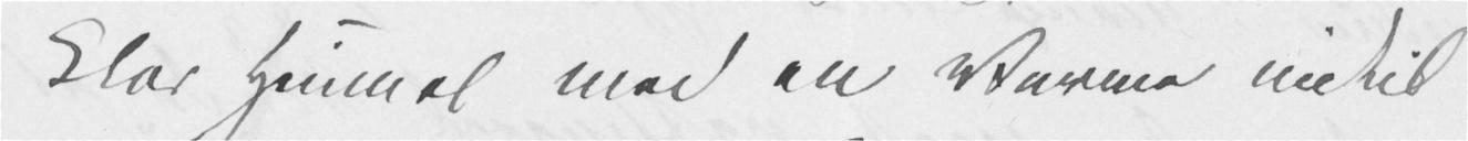klar Himmel med en Varme indtil
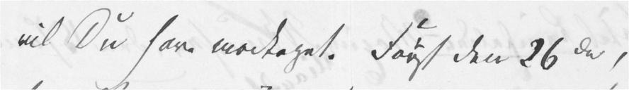vil Du have modtaget. Først den 26de,
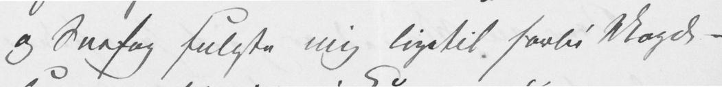og Snefog fulgte mig ligetil forbi Magde-
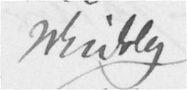Middag
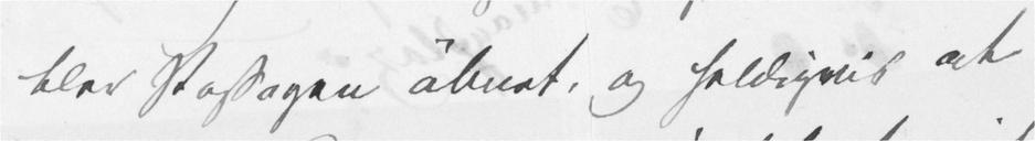blev Passagen åbnet, og heldigvis at
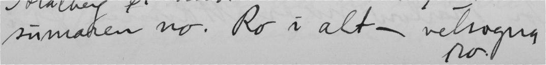sumaren no. Ro i alt – velsogna ro.
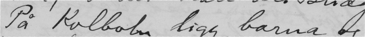På Kolbotn ligg barna og
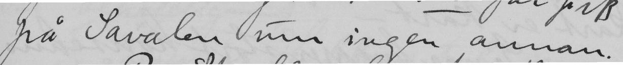på Savalen sum ingen annan.
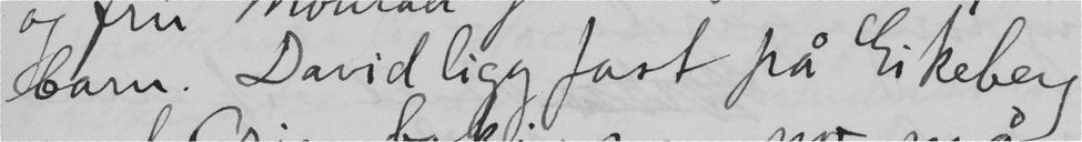barn. David ligg fast på Eikeberg
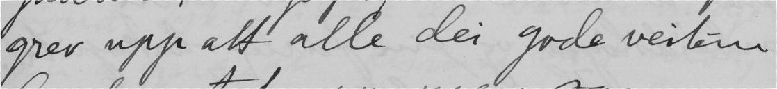grev upp att alle dei gode veilum
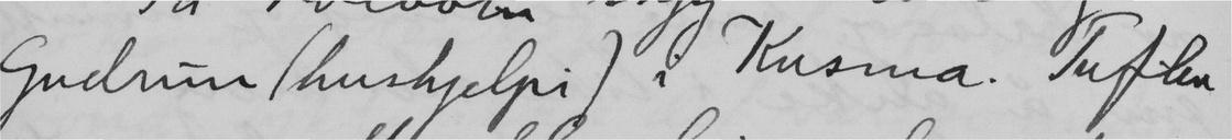Gudrun (hushjelpi) i kusma. Tuften
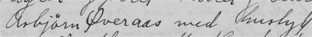Asbjörn Øveraas med huslyd
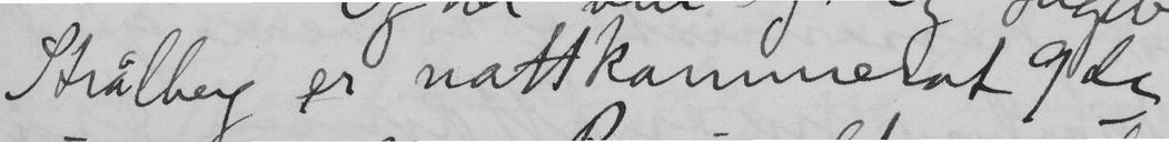Strålberg er nattkammerat 9de
